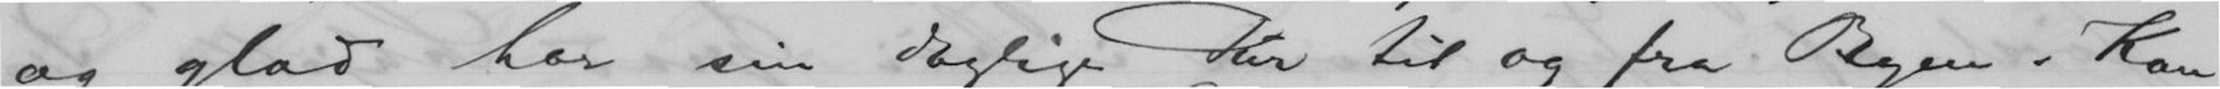og glad har sin daglige Tur til og fra Byen. Kan
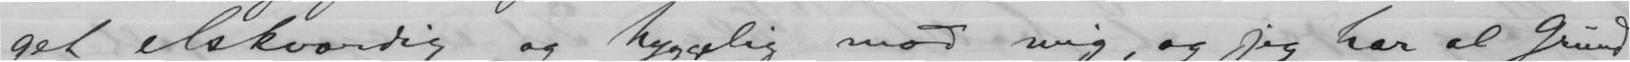get elskværdig og Hyggelig mod mig, og jeg har al Grund
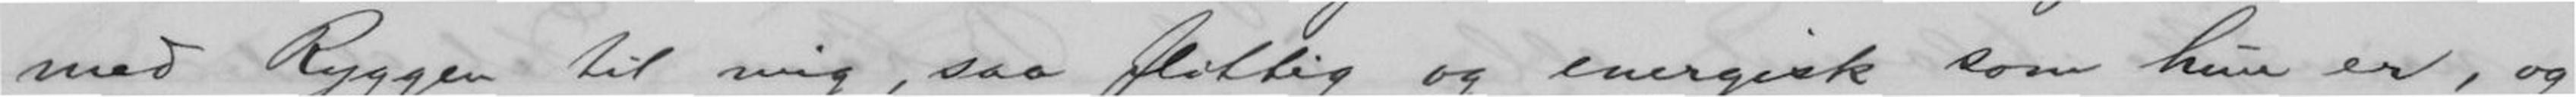med Ryggen til mig, saa flittig og energisk som hun er, og
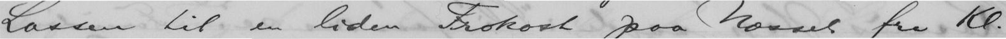Lassen til en liden Frokost paa Næsset fra Kl.
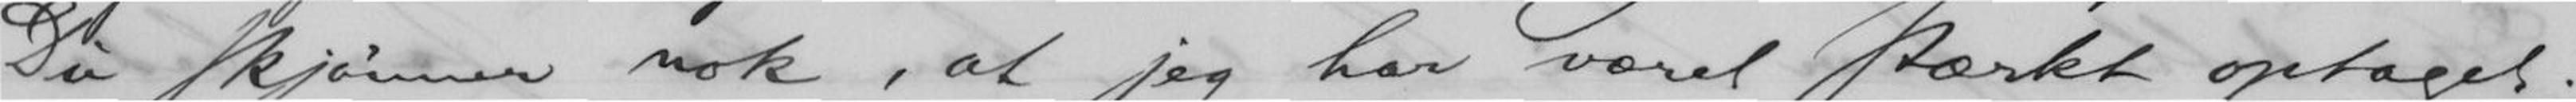Du skjønner nok, at jeg har været stærkt optaget.
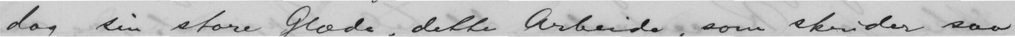dog sin store Glæde, dette Ardeide, som skrider saa
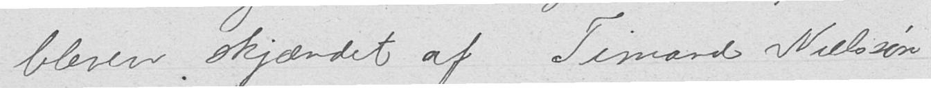bleven skjændet af Timard Nielssøn
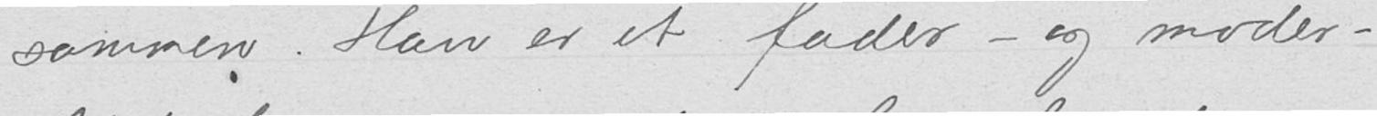sammen.  Han er et fader- og moder-
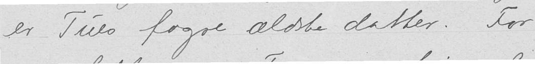er Tues fagre ældste datter. For
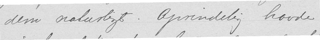dem naturligt. Oprindelig havde
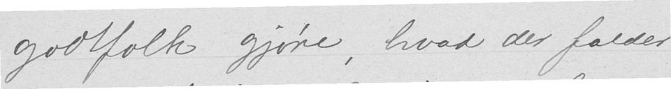godtfolks gjøre, hvad der falder
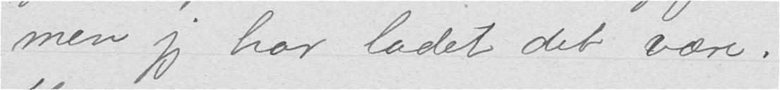men jeg har ladet det være.
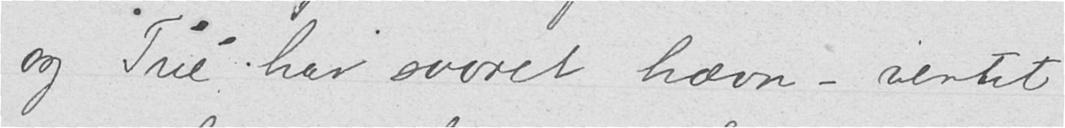og Tue har svoret hævn - ventet
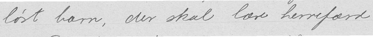løst barn, der skal lære herrefærd
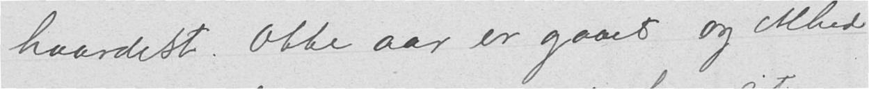haardest. Otte aar er gaaet og Alhed
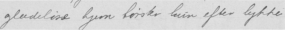glædeløse hjem tørster hun efter lykke
